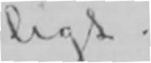ligt.
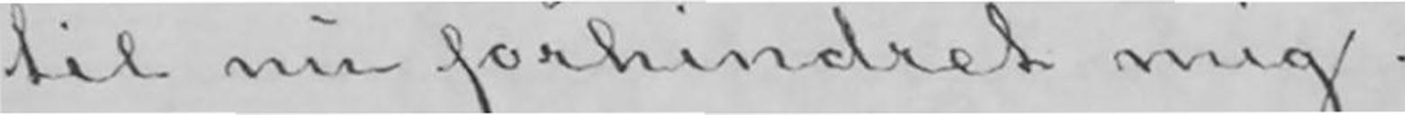til nu forhindret mig.
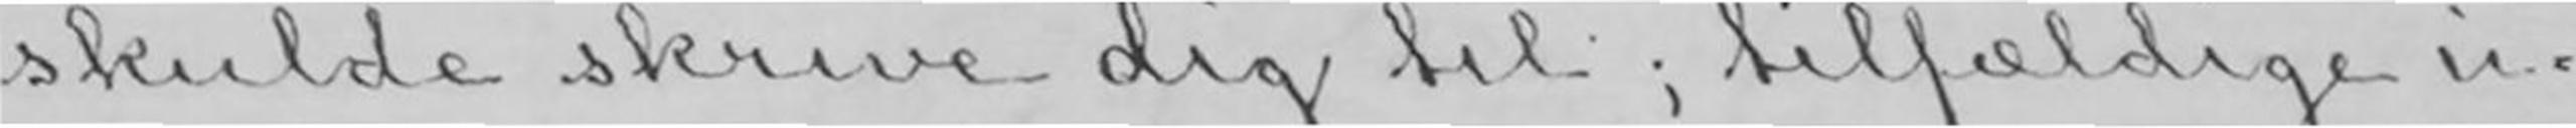skulde skrive dig til; tilfældige u-
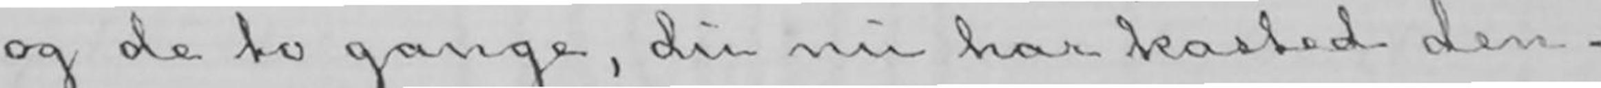og de to gange, du nu har kastet den-
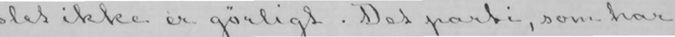slet ikke er gørligt. Det parti, som har
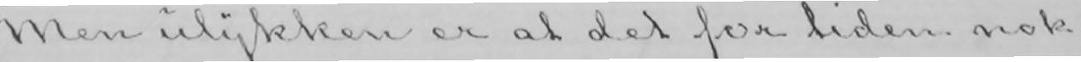Men ulykken er at det for tiden nok
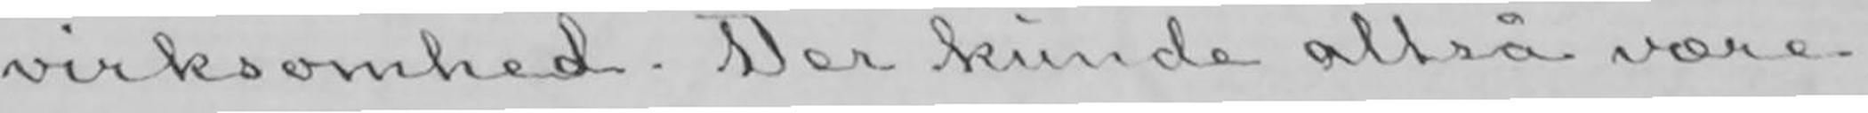virksomhed. Der kunde altså være
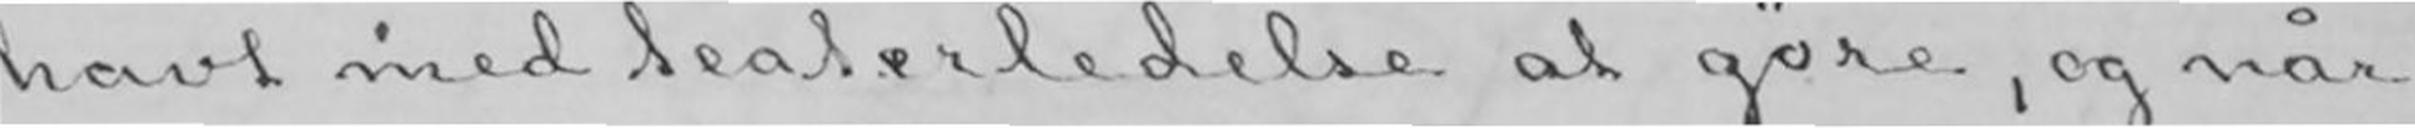havt med teaterledelse at gøre, og når
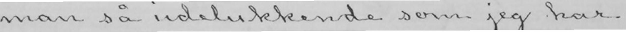man så udelukkende som jeg har
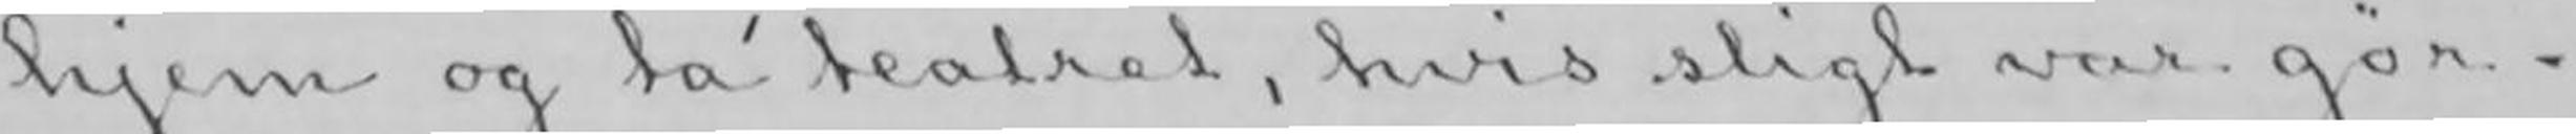hjem og ta´ teatret, hvis sligt var gør-
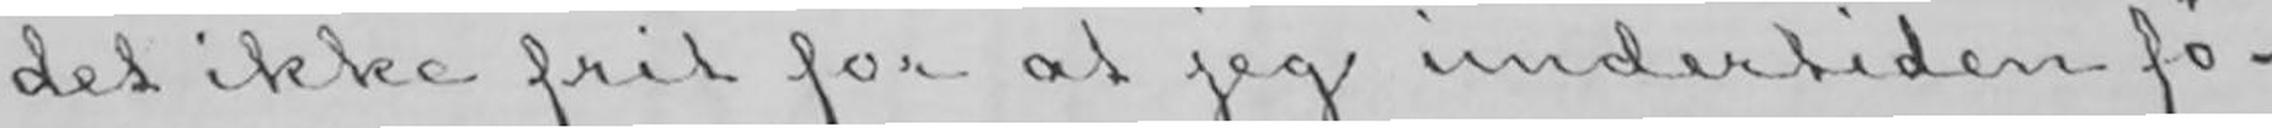det ikke frit for at jeg undertiden fø-
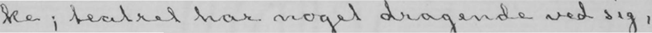ke; teatret har noget dragende ved sig,
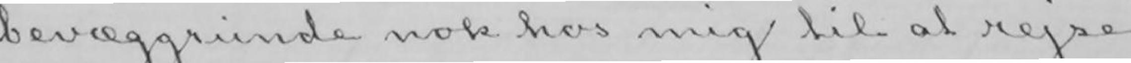bevæggrunde nok hos mig til at rejse
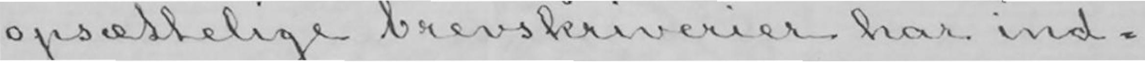opsættelige brevskriverier har ind-
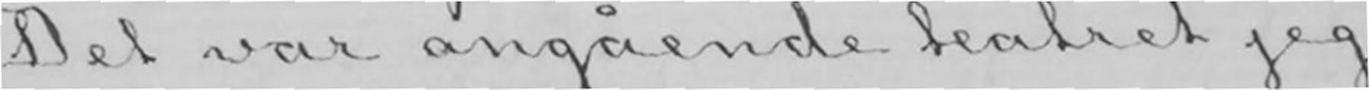Det var angående teatret jeg
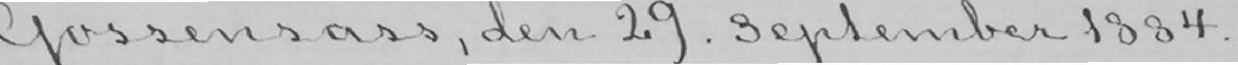Gossensass, den 29. september 1884.
